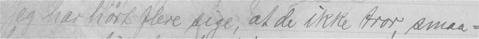Jeg har hørt flere sige, at de ikke tror, smaa-
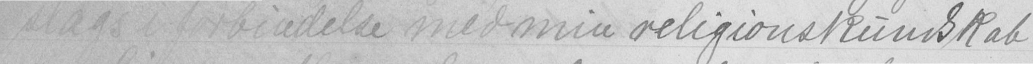slags i forbindelse med min religionskundskab
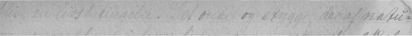bli en livsbetingelse. Det onde og stygge, der af natu-
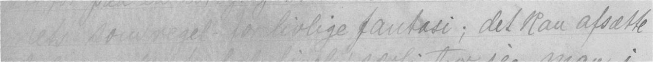nets som regel for livlige fantasi; det kan afsætte
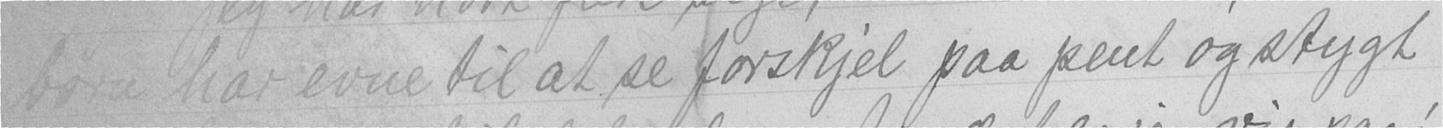børn har evne til at se forskjel paa pent og stygt
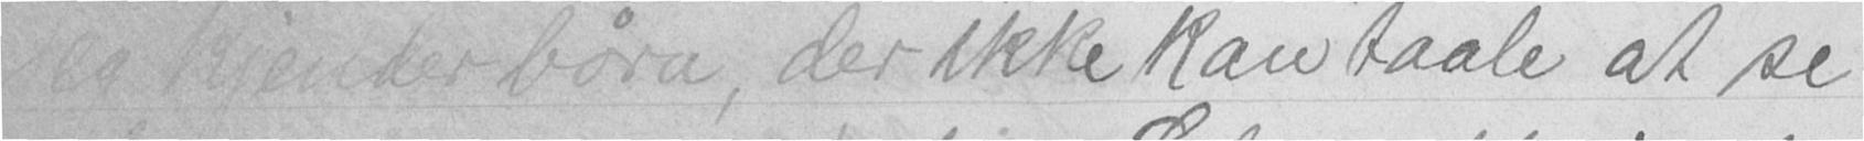Jeg kjender børn, der ikke kan taale at se
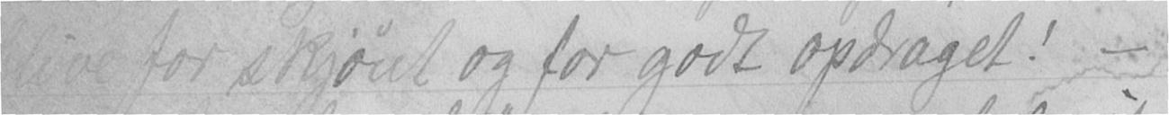blive for skjønt og for godt opdraget! -
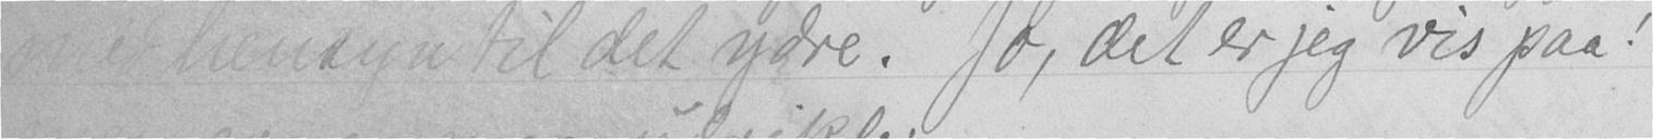med hensyn til det ydre. Jo, det er jeg vis paa!
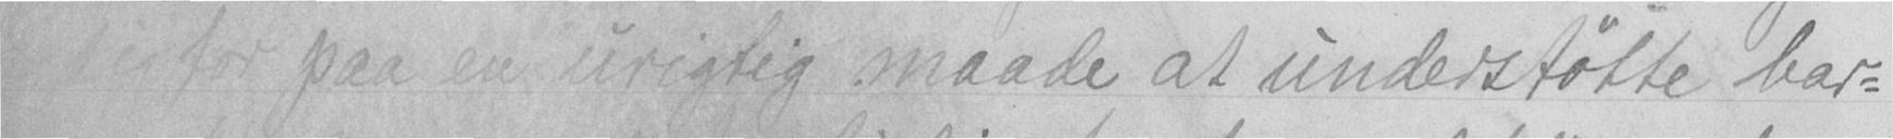sig for paa en urigtig maade at understøtte bar-
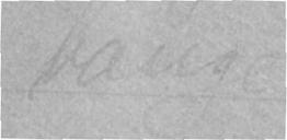bange.
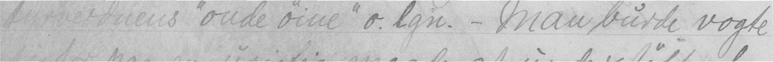tyrverdnens "onde øine" o. lgn. - Man burde vogte
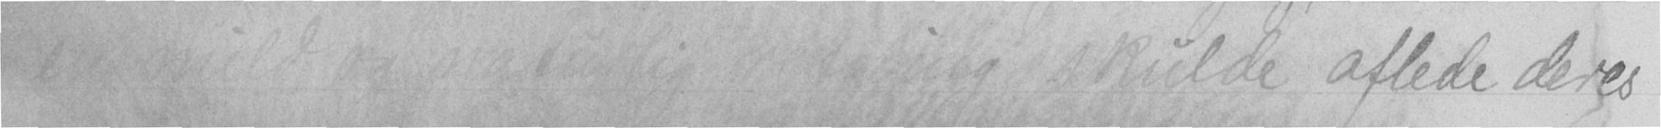mild og  skulde aflede deres
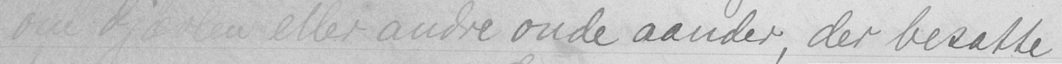om djævlen eller andre onde aander, der besatte
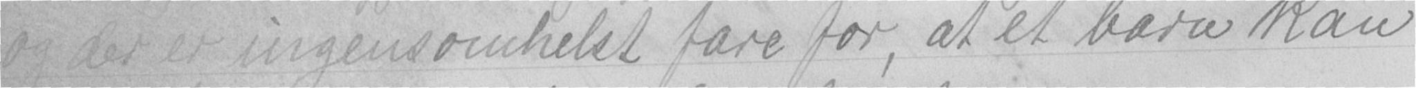og der er ingensomhelst fare for, at et barn kan
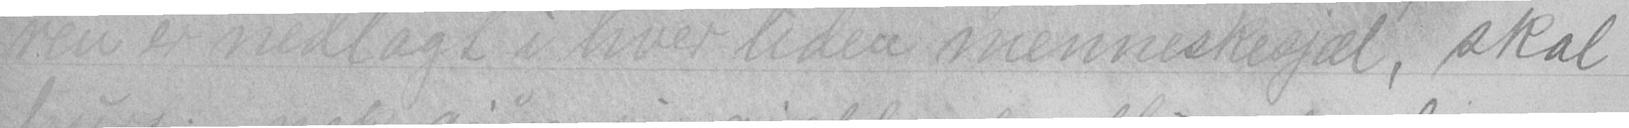ren er nedlagt i hver liden menneskesjæl, skal
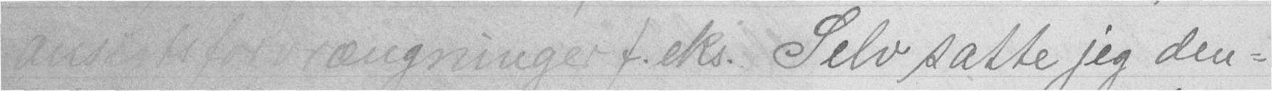ansiktsforvrængninger f.eks. Selv satte jeg den-
